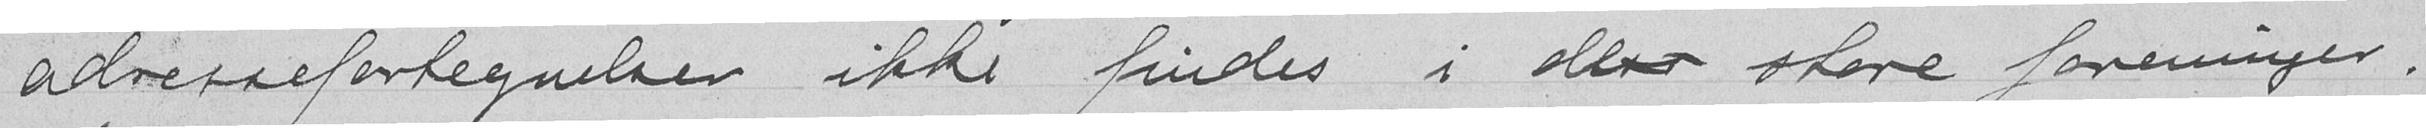adressefortegnelser ikke findes i de store foreninger.
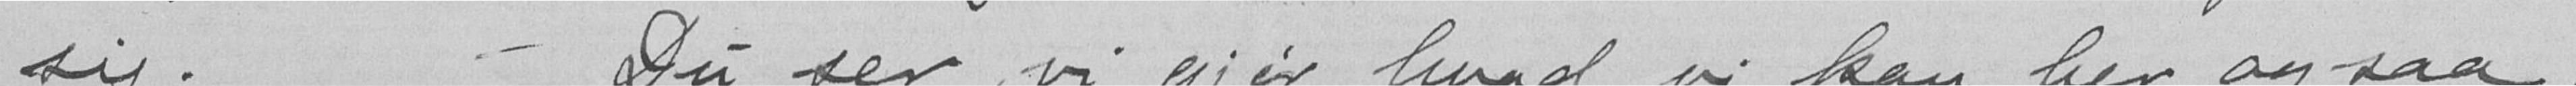sig.- Du ser, vi gjør hvad vi kan her ogsaa
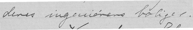deres ingeniørers boliger.
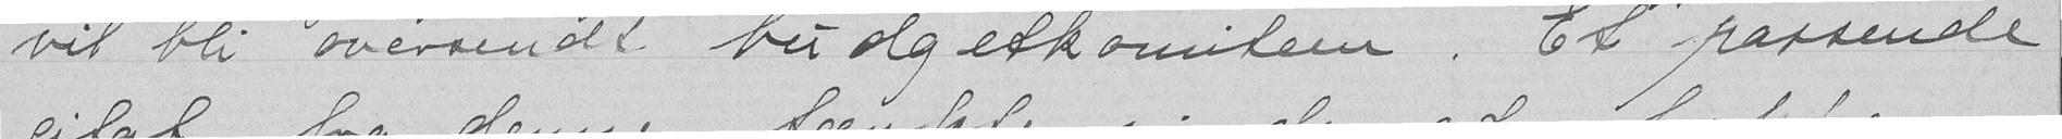vil bli oversendt budgetkomiteen. Et passende
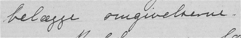belægge omgivelserne.
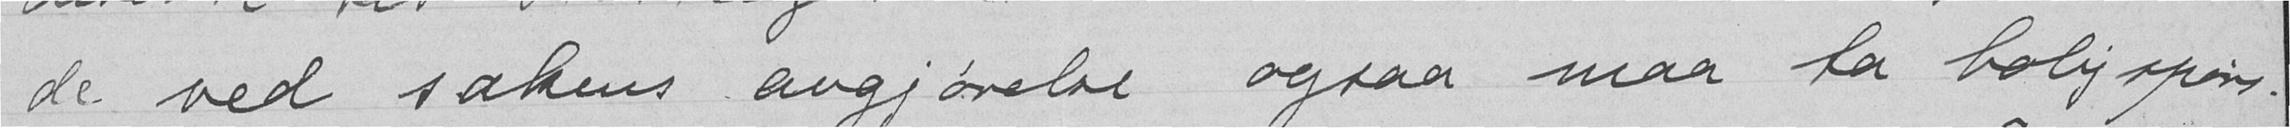de ved sakens angjørelse ogsaa maa fa boligspørs-
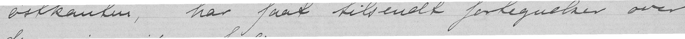østkanten, har faat tilsendt fortegnelser over
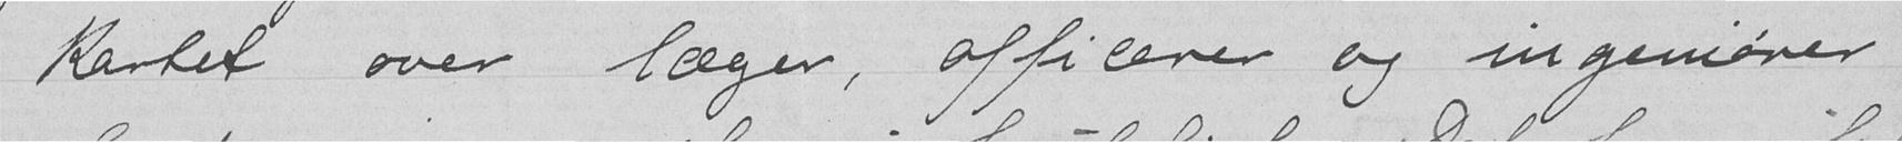Kartet over læger, officerer og ingeniører
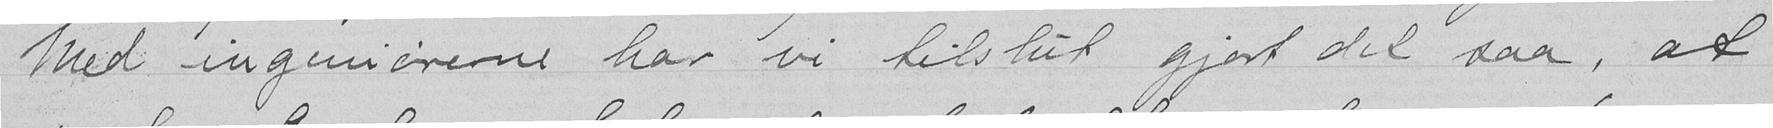Med ingeniørerne har vi tilslut gjort det saa, at
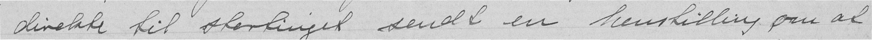direkte til stortinget sendt en henstilling om at
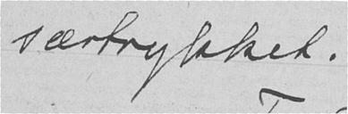særtrykket.
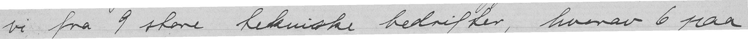vi fra 9 store tekniske bedrifter, hvorav 6 paa
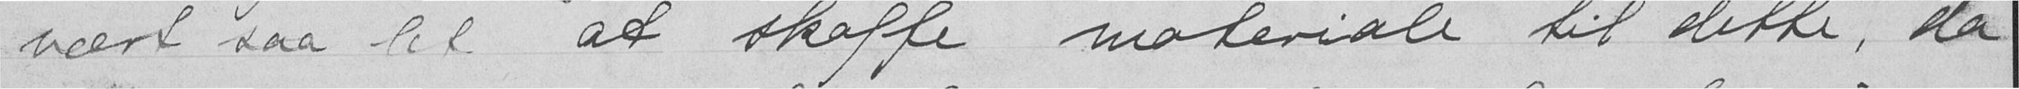vært saa let at skaffe materiale til dette, da
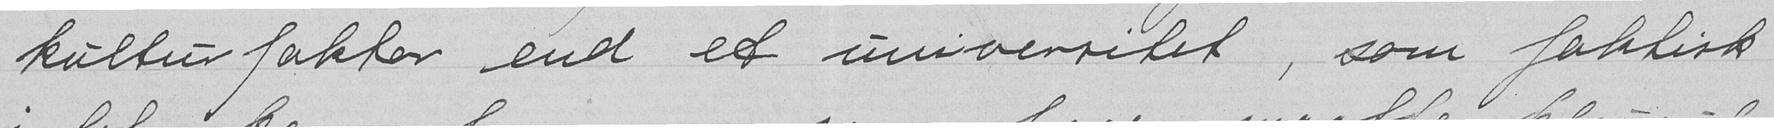kulturfaktor end et universitet, som faktisk
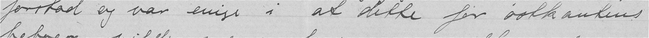forstod og var enige i at dette for østkantens
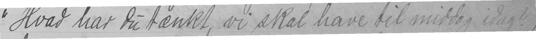"Hvad har du tænkt, vi skal have til middag idag?
